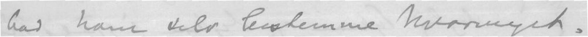lad ham selv bestemme hvormeget.
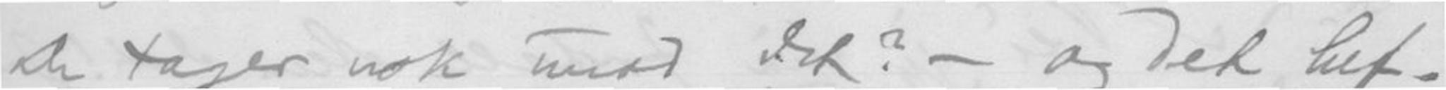De tager nok imod det? - og det hef-
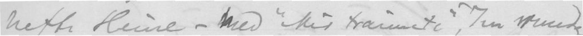hefte Heine - Med "Mir träumte", "Im wunder-
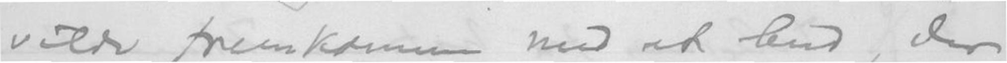vilde fremkomme med et bud, der
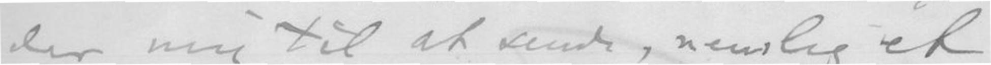der mig til at sende, nemlig et
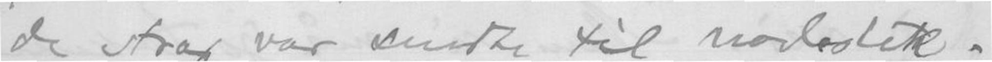de strax var sendte til nodestik-
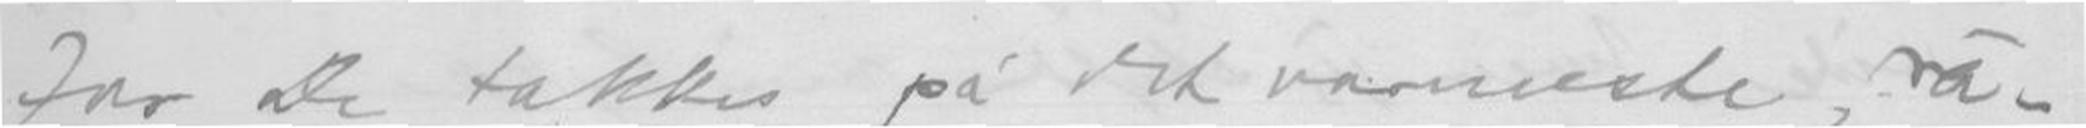for De takkes på det varmeste, rå-
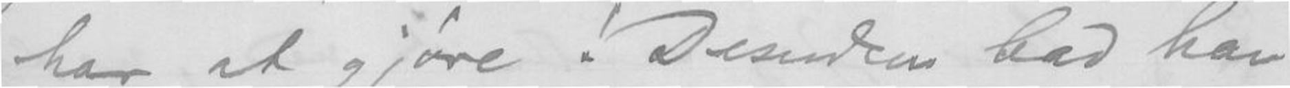har at gjøre! Desuden lod han
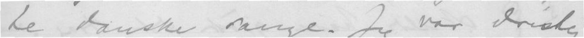te danske sange. Jeg var dristig
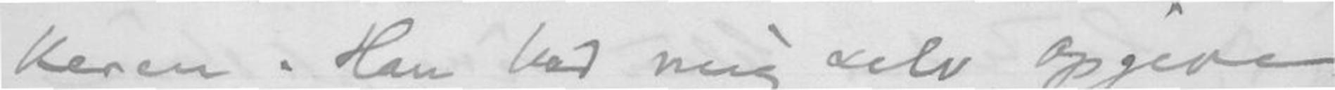keren. Han lod mig selv opgive
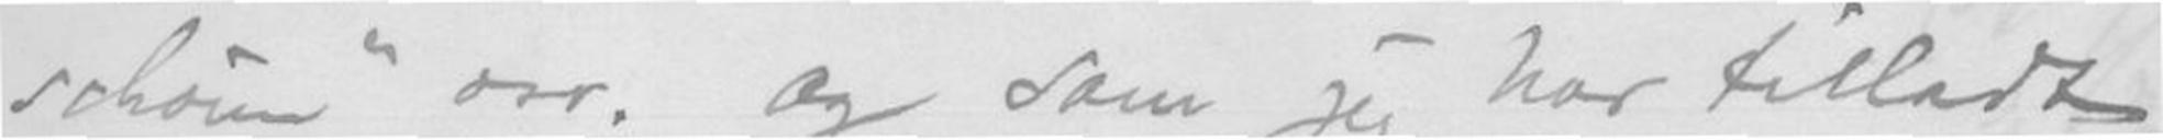schönen" osv. Og som jeg har tilladt
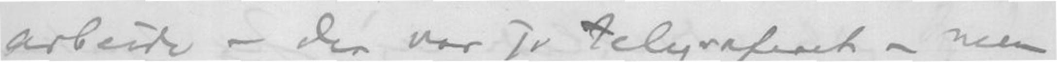arbeide - der var jo telegraferet - men
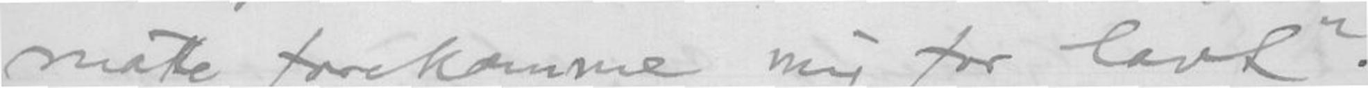måtte forekomme mig for lavt".
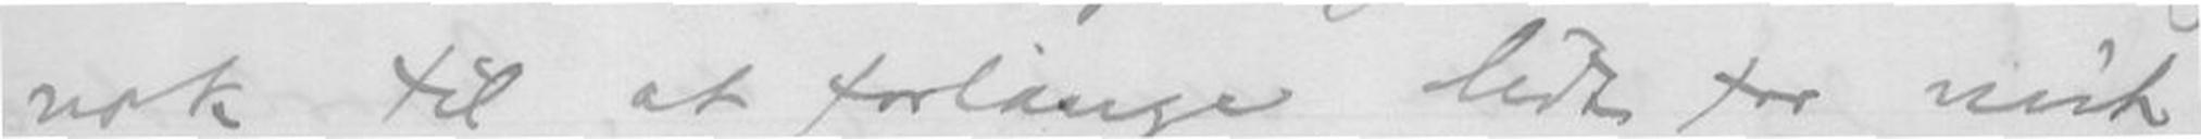nok til at forlange lidt for mit
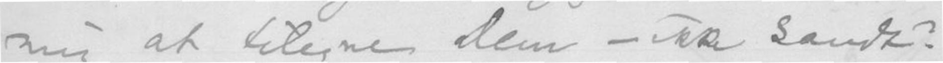mig at tilegne Dem - ikke sandt?
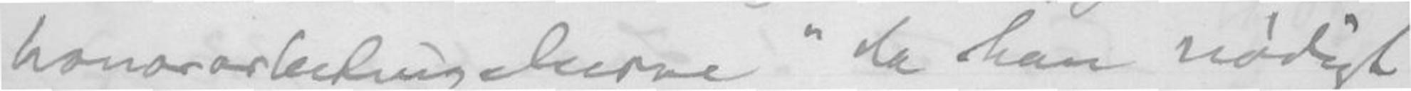honorarbetingelserne "da han nødigt
